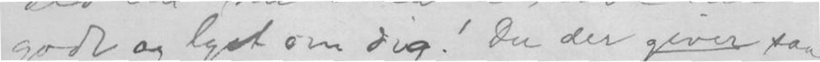godt og lyst om dig! Du der giver saa
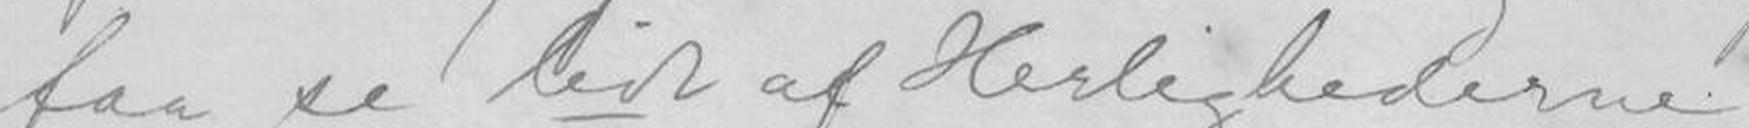faa se lidt af Herlighederne
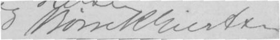Børre R Giertsen
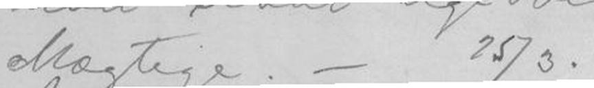Mægtige. -25/3.
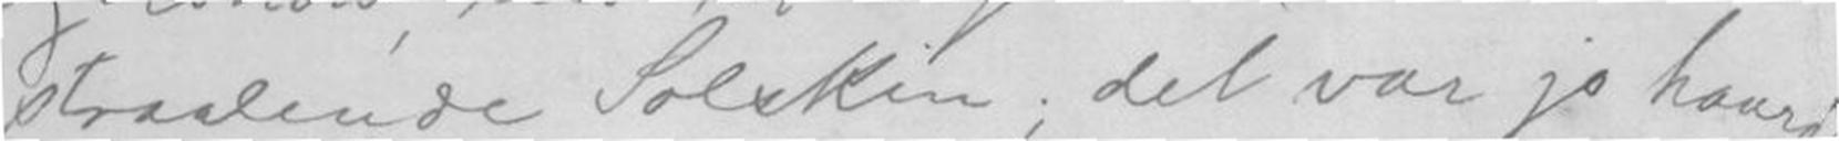straalende Solskin; det var jo haardt
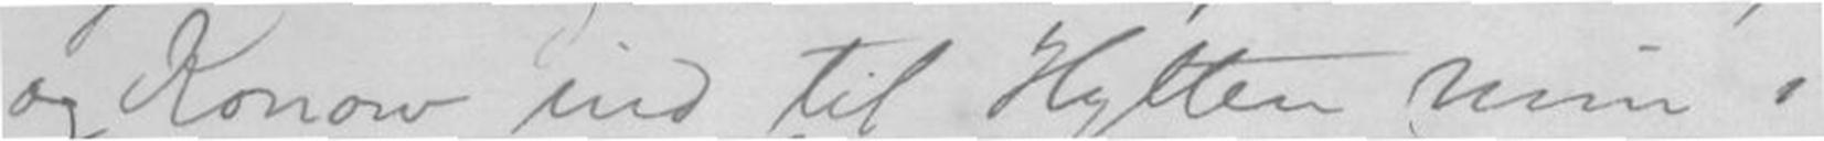og Konow, ind til Hytten min i
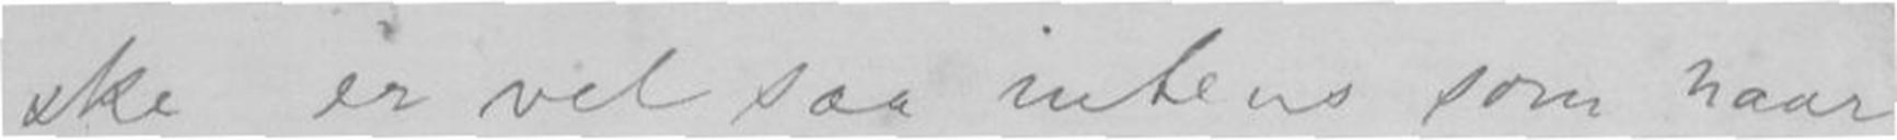ske er vel saa intens som naar
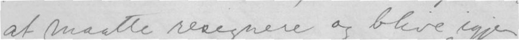at maatte resignere og blive igjen
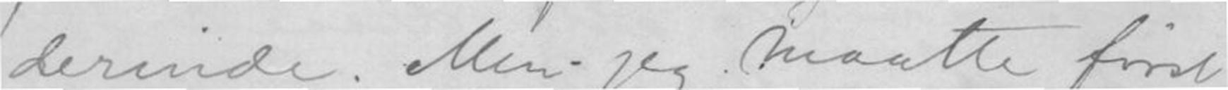derinde. Men jeg maatte først
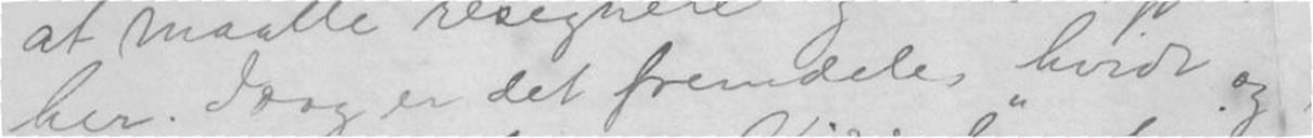her. Idag er det fremdeles hvidt og
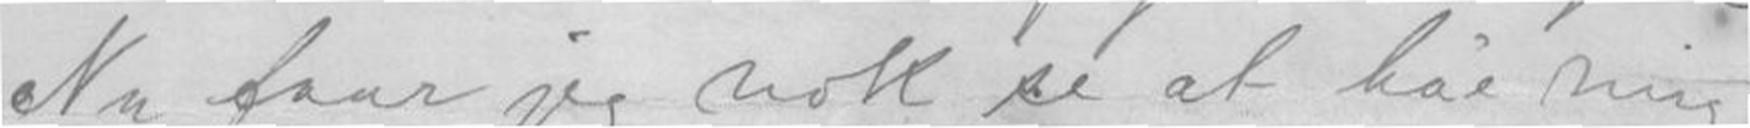Nu faar jeg nok se at ha'e mig
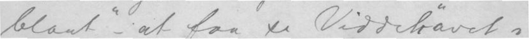blaat - at faa se Viddehavet i
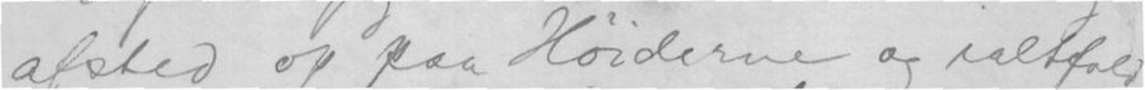afsted op paa Høiderne og ialtfald
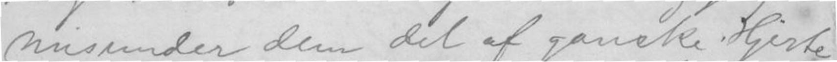misunder dem det af ganske Hjerte
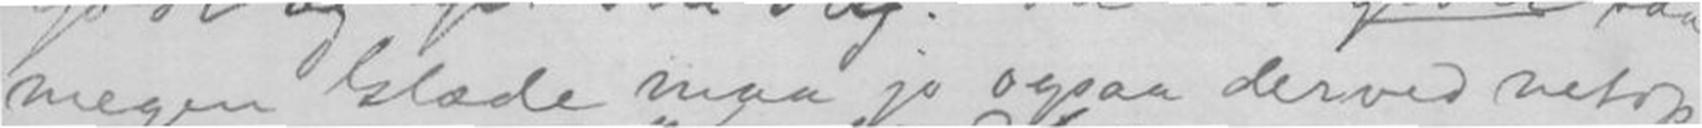megen Glæde maa jo ogsaa derved netop
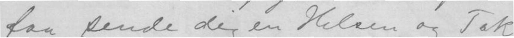faa sende dig en Hilsen og Tak
